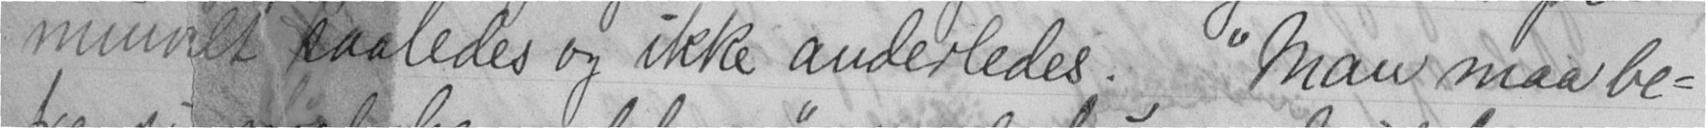mnin et saaledes og ikke anderledes.  "Man maa be-
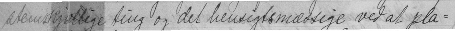stem kjellige ting og det hensigtsmæssige ved at pla-
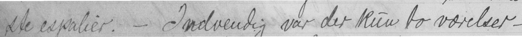ste espalier. - Indvendig var der kun to værelser -
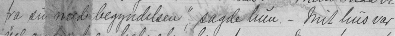fra si med begyndelsen", - sagde hun. - Mit hus var
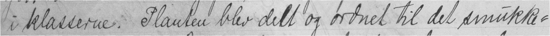i klasserne. Planten blev delt og ordnet til det smukke-
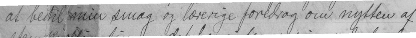at be til min smag og lærerige foredrag om nytten af
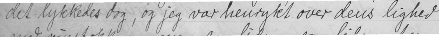det lykkedes dog, og jeg var henrykt over dens lighed
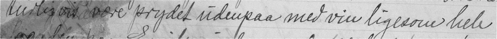turligvis være prydet udenpaa med vin ligesom hele
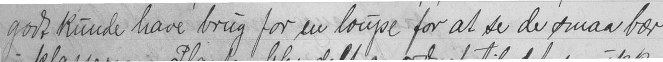godt kunde have brug for en loupe for at se de smaa bær
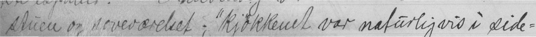stuen og soveværelset; "kjøkkenet var naturligvis i side-
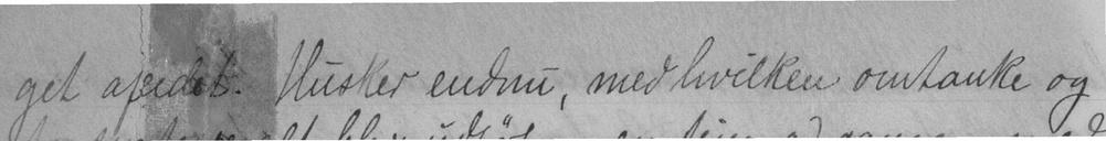get af eidet. Husker endnu, med hvilken omtanke og
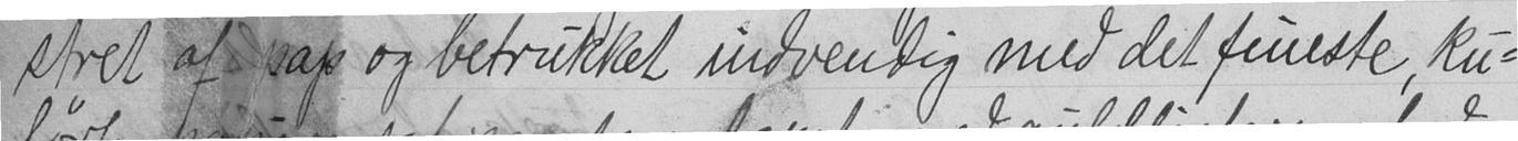stret af pap og betrukket indvendig med det fineste ku-
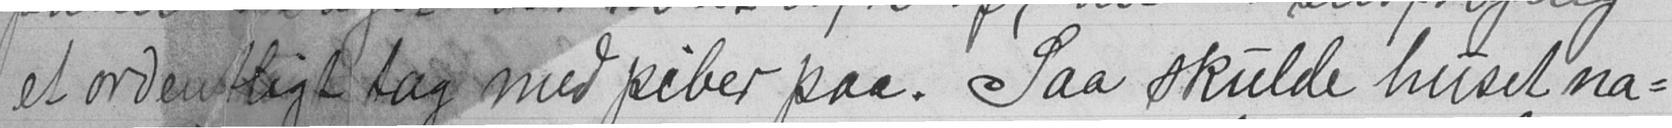et ordentligt tag med piber paa. Saa skulde huset na-
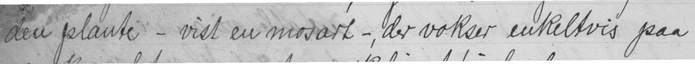den plante - vist en mosart -, der vokser enkeltvis paa
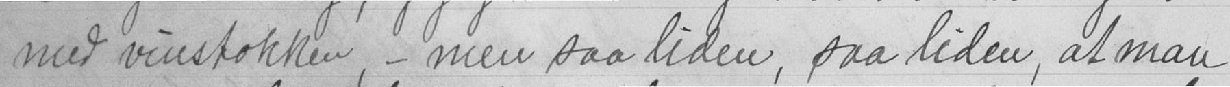med vinstokken, - men saa liden, saa liden, at man
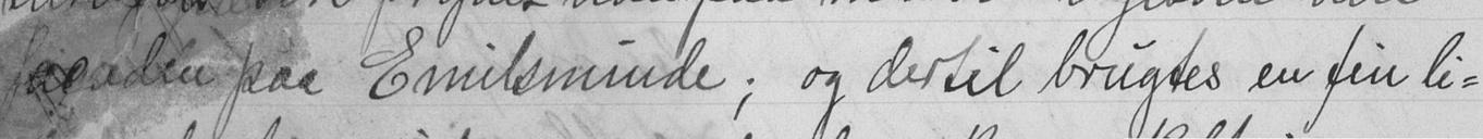facaden paa Emilsminde; og dertil brugtes en fin li-
